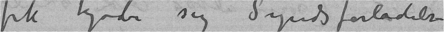fik kjøbe sig Syndsforladelse
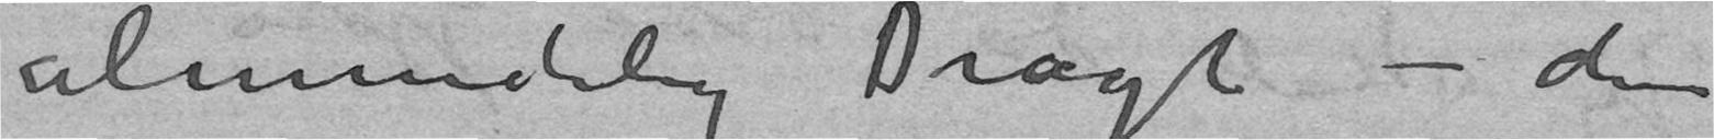almindelig Dragt – den
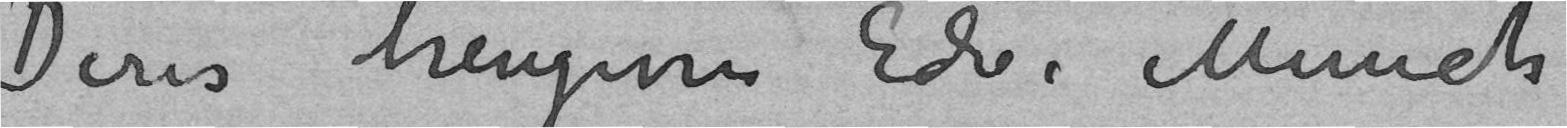Deres hengivne Edv. Munch
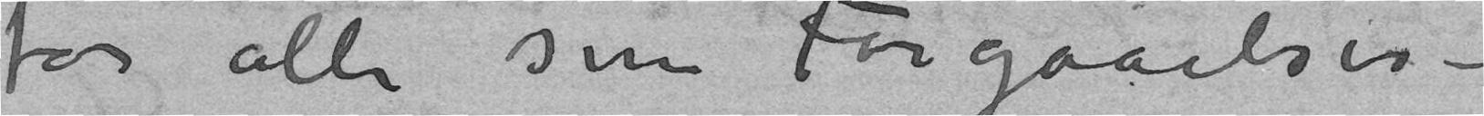for alle sine Forgaaelser –
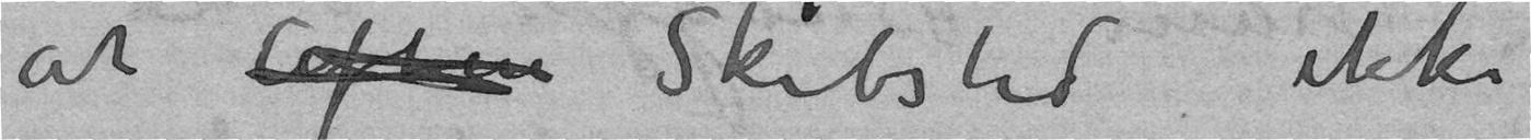at Aften Skibsted ikke
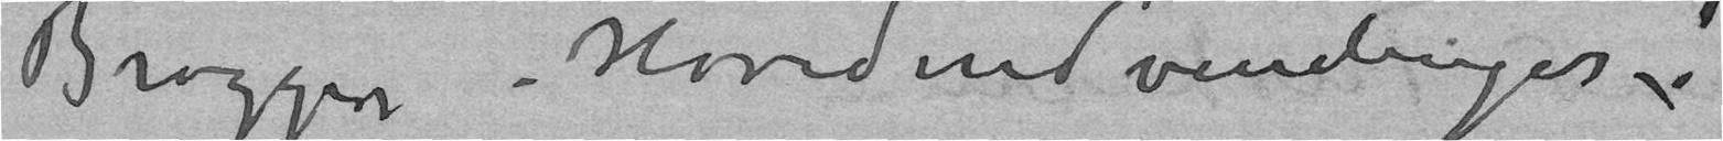var Brøggers Hovenindvendinger!
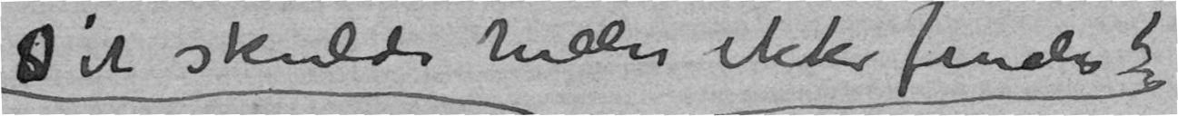Det skulde heller ikke findes
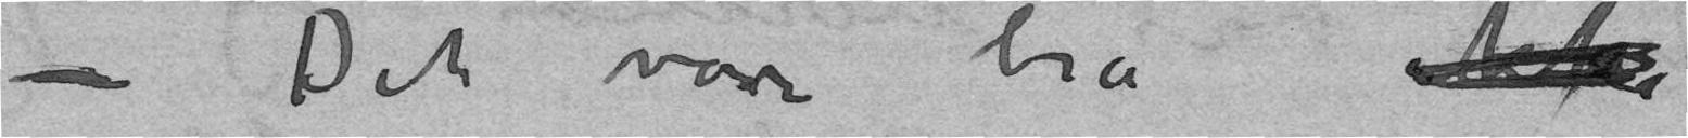– Det var bra ikke
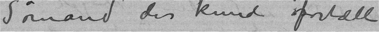Sømand der kunde fortælle
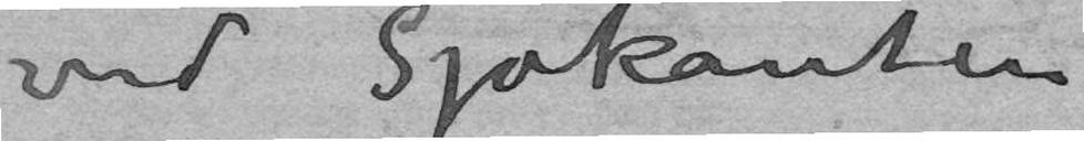ved Sjøkanten
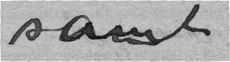samt
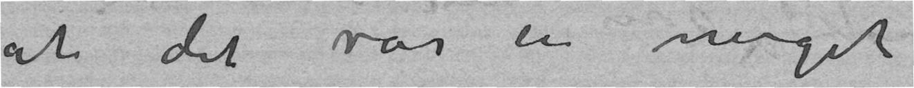at det var en meget
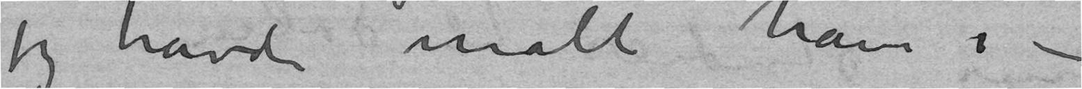jeg havde malt ham i –
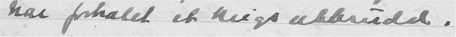har forhalet et krigsutbrudd.
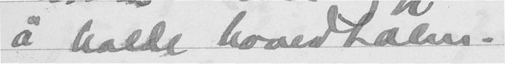i holde hovedtalen.
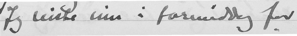Jeg reiste inn i formiddag for
